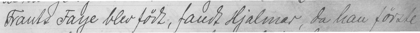Frants Faye blev født, fandt Hjalmar, da han første
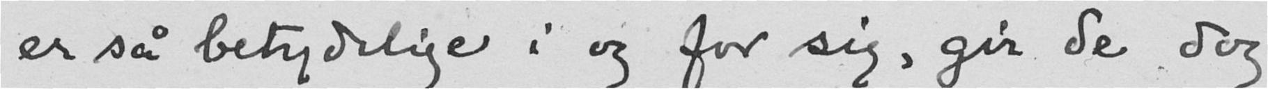er så betydelige i og for sig, gir de dog
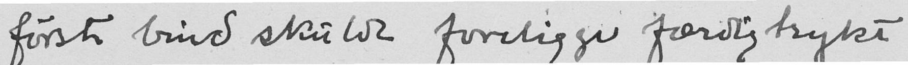første bind skulde foreligge færdigtrykt
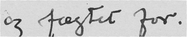og fægtet for.
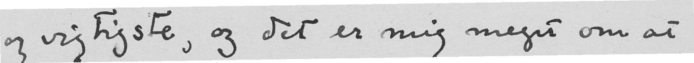og vigtigste, og det er mig meget om at
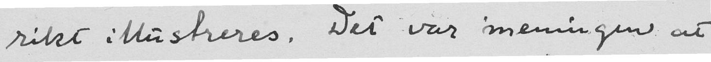rikt illustreres. Det var meningen at
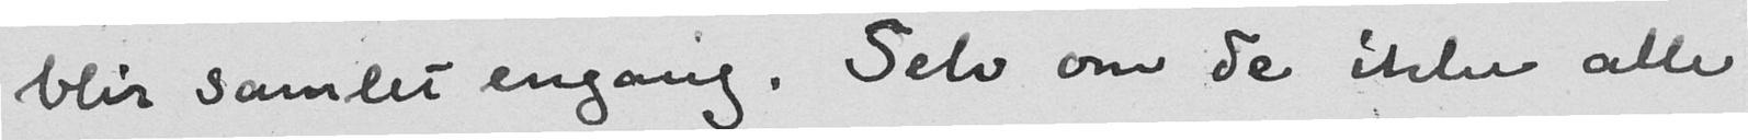blir samlet engang. Selv om de ikke alle
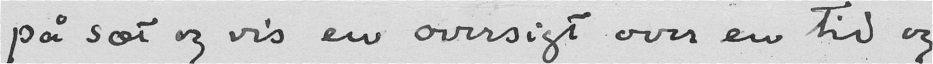på sæt og vis en oversigt over en tid og
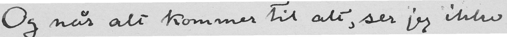Og når alt kommer til alt, ser jeg ikke
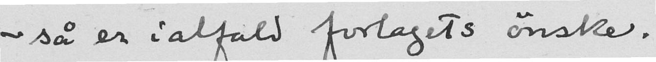- så er ialfald forlagets ønske.
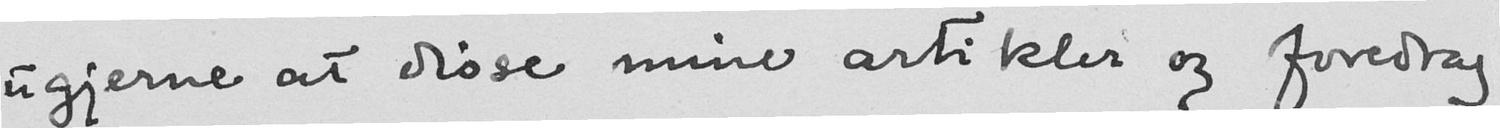ugjerne at disse mine artikler og foredrag
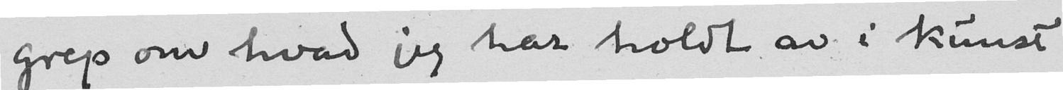grep om hvad vi har holdt av i kunst
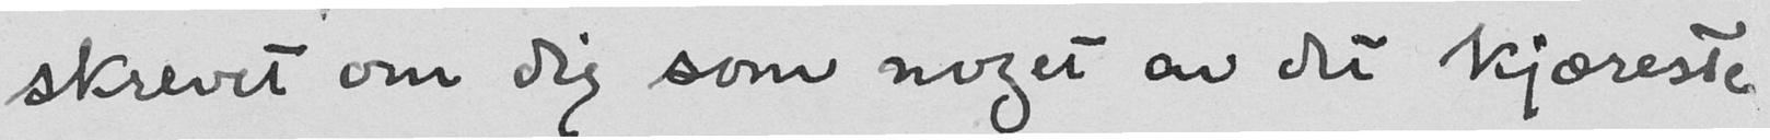skrevet om dig som noget av det kjæreste
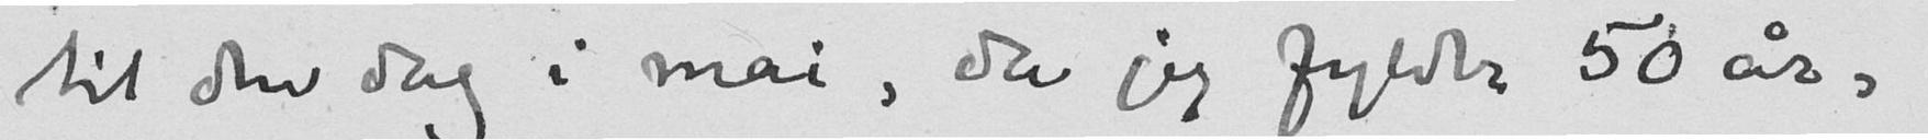til den dag i mai, da jeg fylder 50 år,
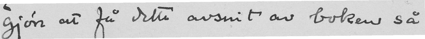gjøre at få dette avsnit av boken så
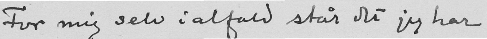For mig selv ialfald står det jeg har
